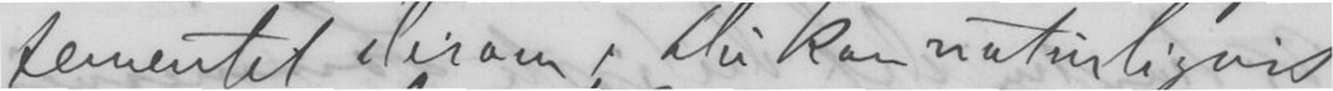tementet derom, Du kan naturligvis
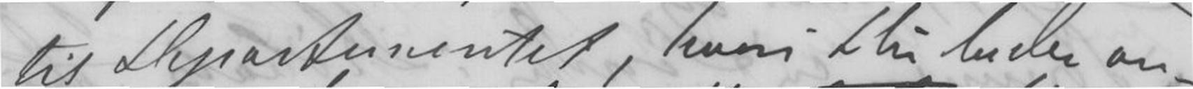til Departementet, hvori Du beder om
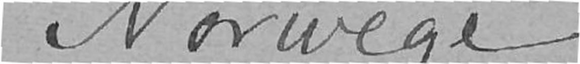Norwège
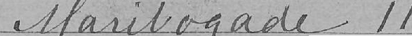Maribogade 11
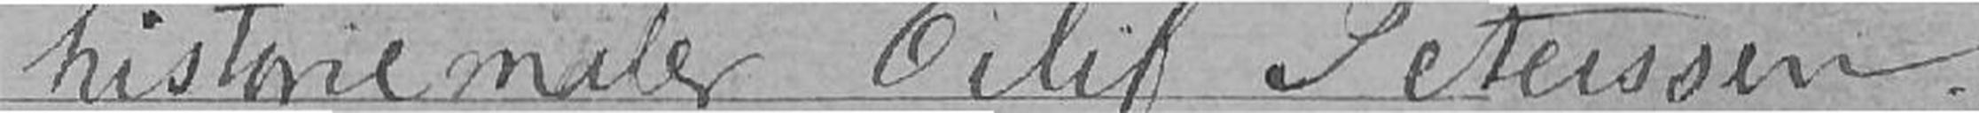historiemaler Eilif Peterssen.
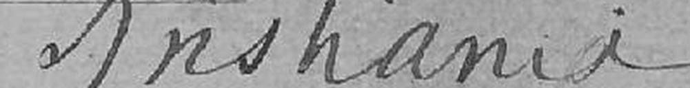Kristiania
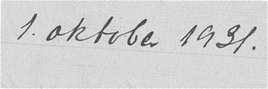1. oktober 1931.
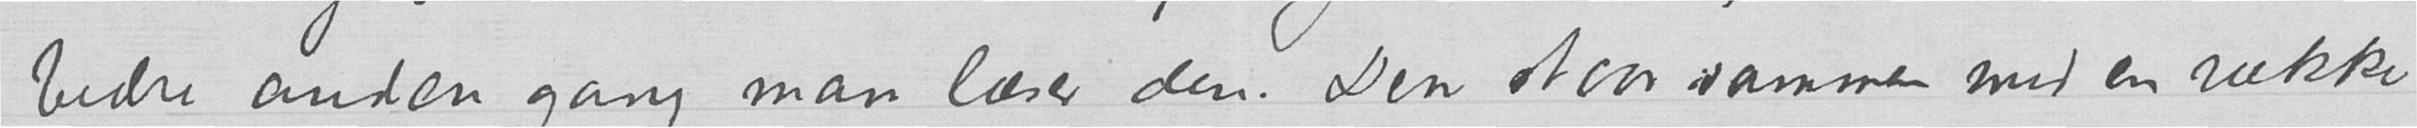bedre anden gang man læser den. Den staar sammen med en række
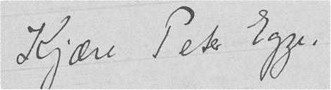Kjære Peter Egge,
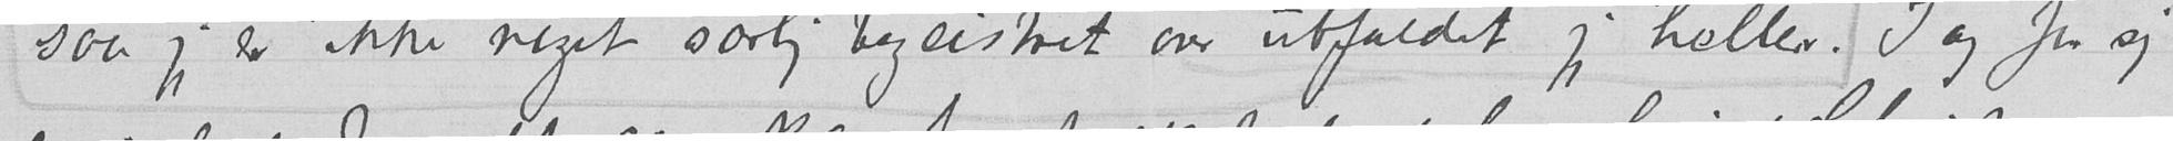saa jeg er ikke noget særlig begeistret over utfaldet jeg heller. I og for sig
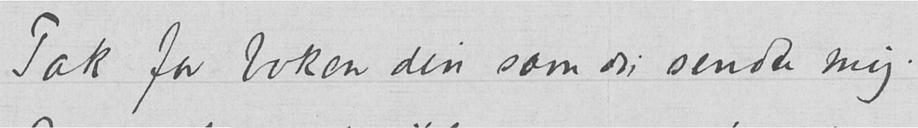Tak for boken din som du sendte mig.
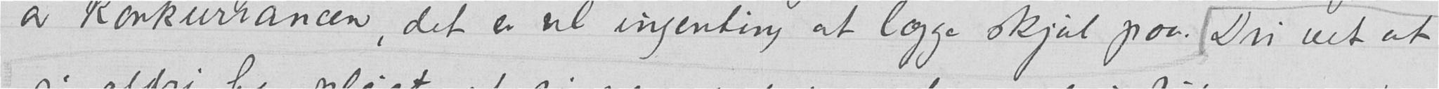av konkurrancen, det er vel ingenting at legge skjul paa. Du vet at
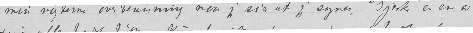min nøgterne overbevisning naar jeg sier at jeg synes, "Gjester" er en av
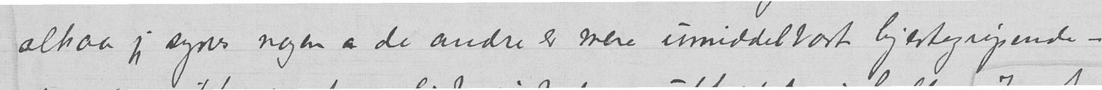altsaa jeg synes nogen av de andre er mere umiddelbart hjertegripende –
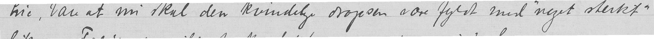Lie, bare at nu skal den kvindelige dropsen være fyldt med "noget sterkt"
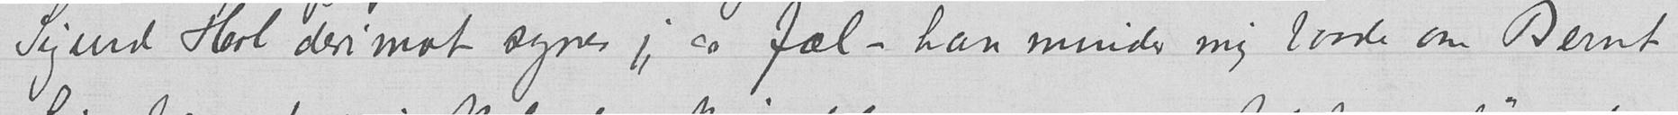Sigurd Hoel derimot synes jeg er fæl – han minder mig baade om Bernt
